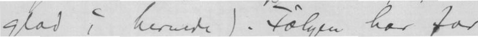glad i hernede). Følgen har for
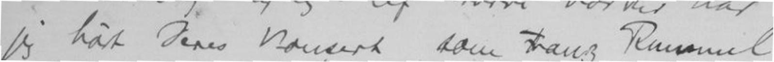jeg hørt Deres konsert, som Franz Rummel
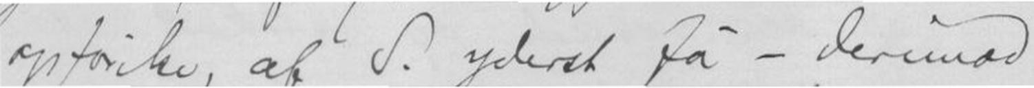opførelse, af S. yderst få - derimod
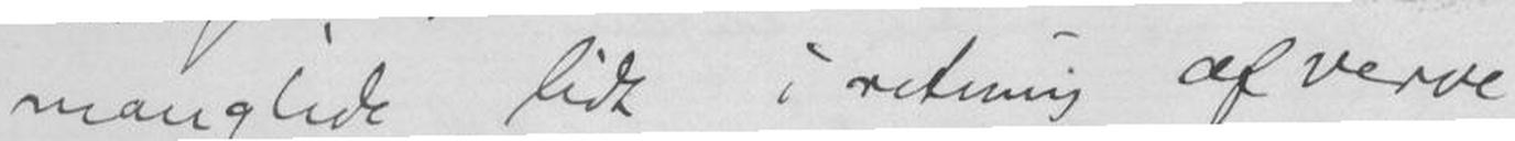manglede lidt i retning af verve
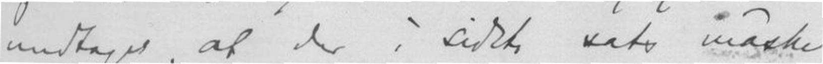undtages, at der i sidste sats måske
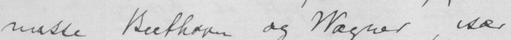masse Beethoven og Wagner, især
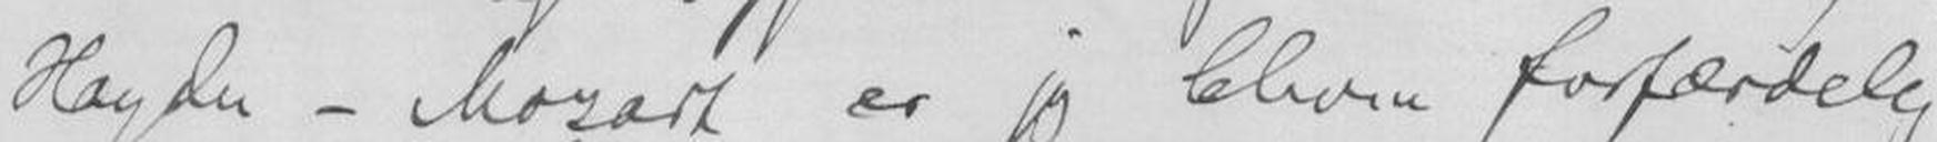Haydn - Mozart er jeg bleven forfærdelig
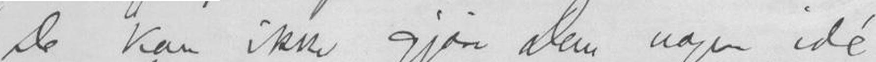De kan ikke gjøre Dem nogen idé
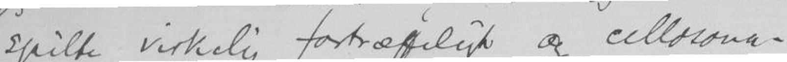spilte virkelig fortræffeligt og cellosona-
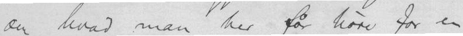om hvad man her får høre for en
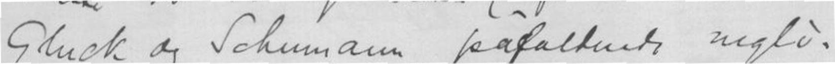Gluck og Schumann påfaldende negli-
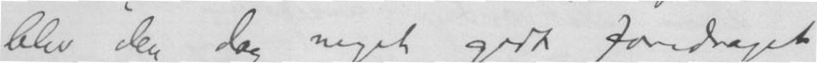blev den dog meget godt foredraget
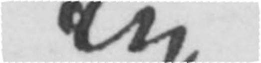en
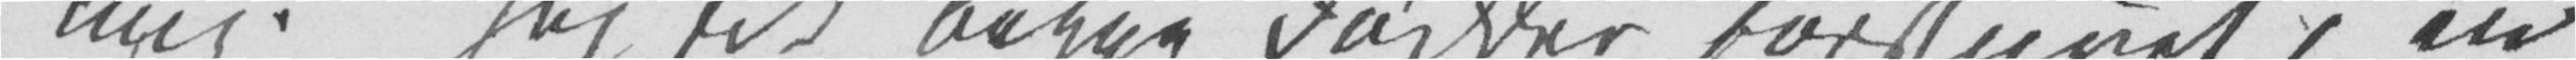mig. Jeg fik begge Fødder forstuvet i en
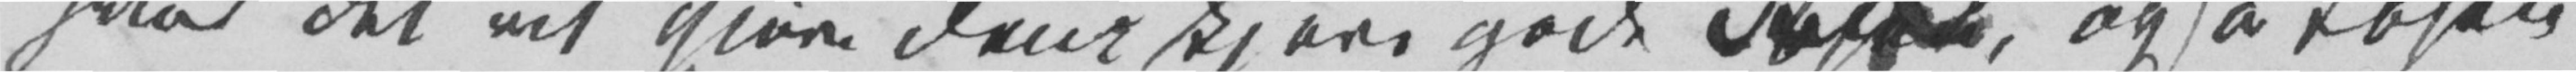hvor det vil gjøre Dem kjere gode Fru I, også Ibsen
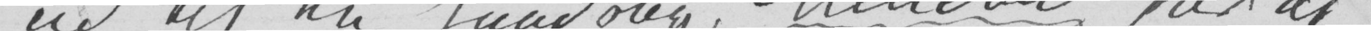ud til en Landsby, Meudon, for at
